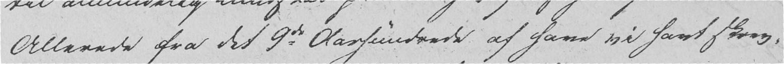Allerede fra det 9de Aarhundrede af have vi havt skrev-
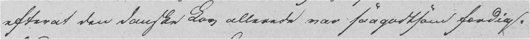efterat den danske Lov allerede var saagodtsom færdig.
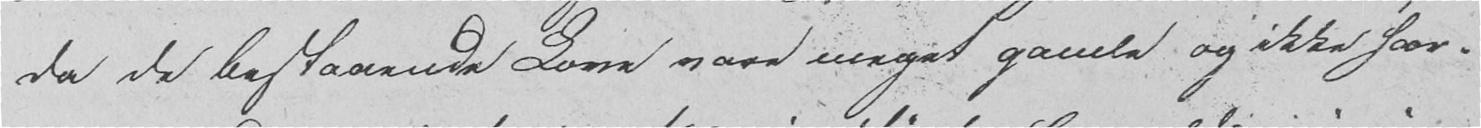da de bestaaende Love vare meget gamle og ikke har-
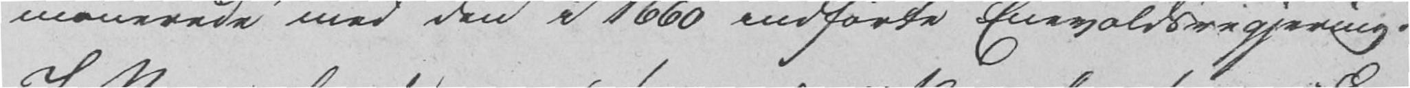monerede med den i 1660 indførte Enevoldsregjering.
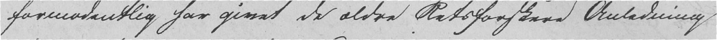formodentlig har givet de ældre Retsforskere Anledning
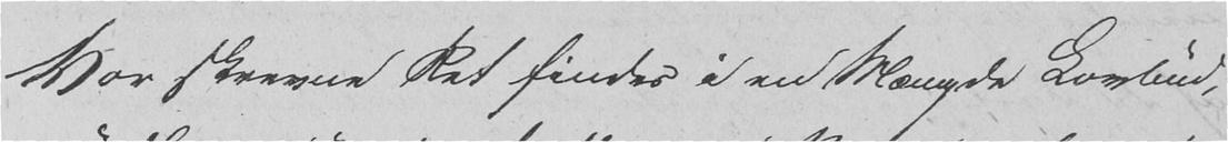Vor skrevne Ret findes i en Mængde Lovbud,
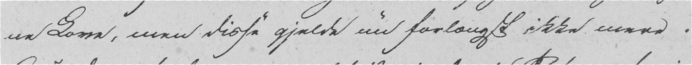ne Love, men disse gjelde nu forlængst ikke mere.
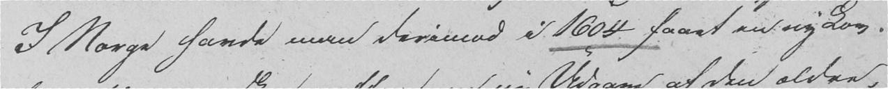I Norge havde man derimod i 1604 faaet en ny Lov-
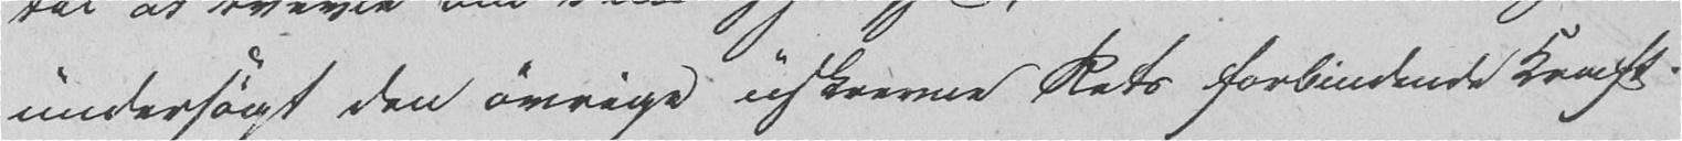undersøgt den øvrige uskrevne Rets forbindende Kraft.
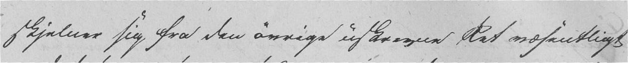skjelner sig fra den øvrige uskrevne Ret væsentligt
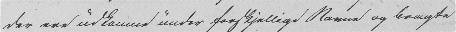der ere udkomne under forskjellige Navne og brugte
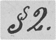§ 2.
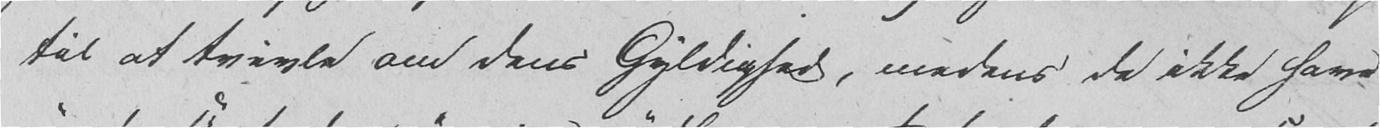til at tvivle om dens Gyldighed, medens de ikke have
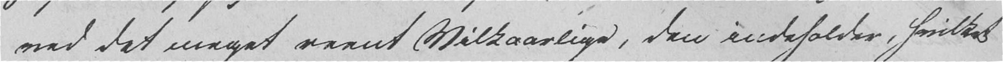ved det meget reent Vilkaarlige, den indeholder, hvilket
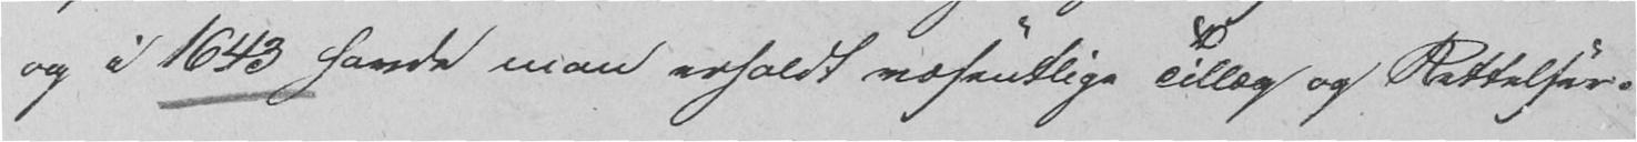og i 1643 havde man erholdt væsentlige Tillæg og Rettelser.
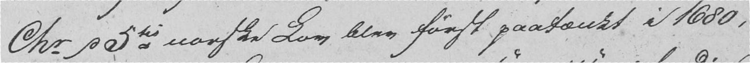Chr d 5tes norske Lov blev først paatænkt i 1680,
